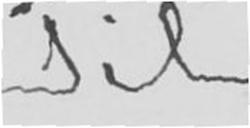Til
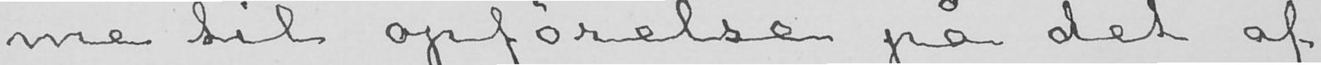me til opførelse på det af
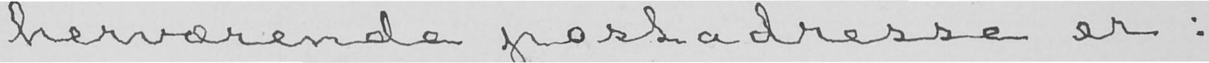herværende postadresse er:
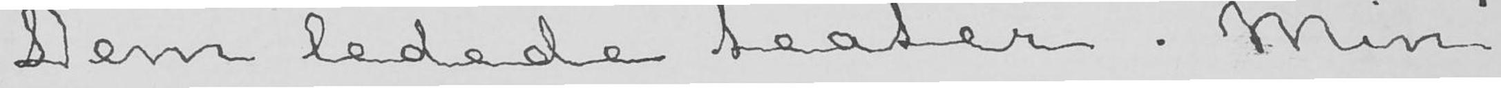Dem ledede teater. Min
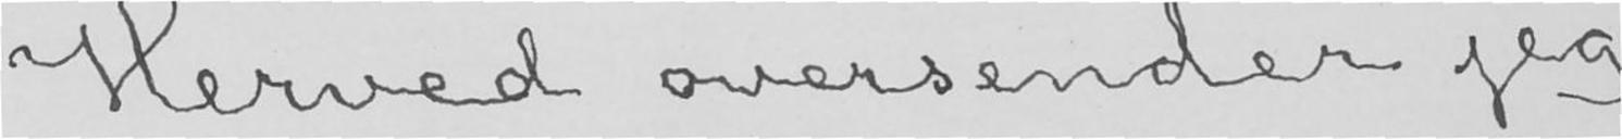Herved oversender jeg
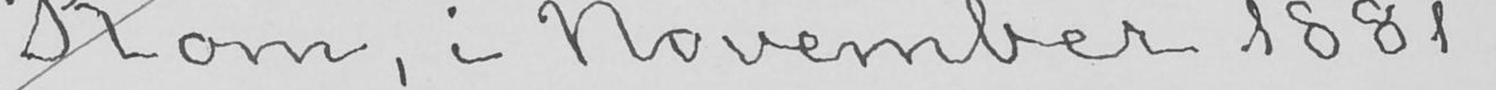Rom, i November 1881.
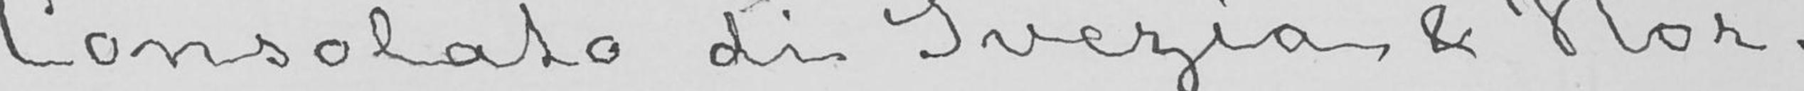Consolato di Svezia & Nor-
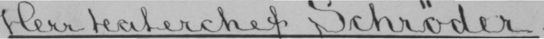Herr teaterchef Schrøder.
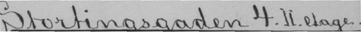Stortingsgaden 4. II. etage.
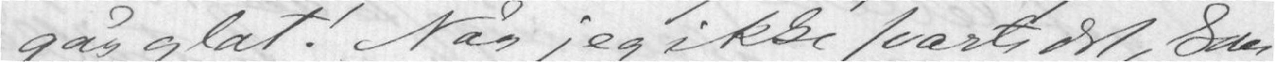går glat! Når jeg ikke svarte det, kom
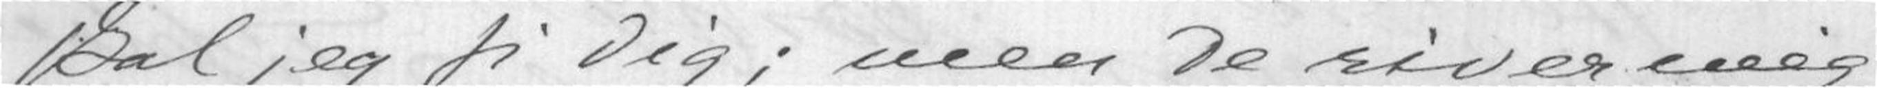skal jeg si dig; men de river mig
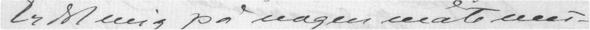Er det mig på nogen måte mu-
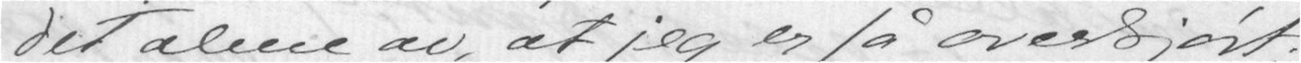det alene av, at jeg er så overkjørt;
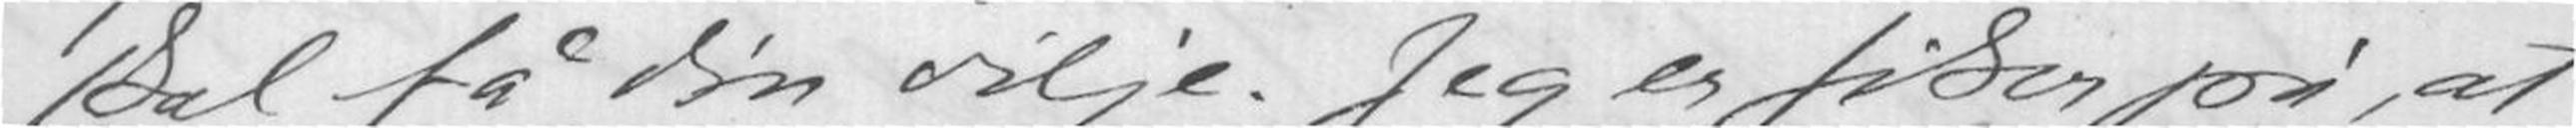skal få din vilje. Jeg er siker på, at
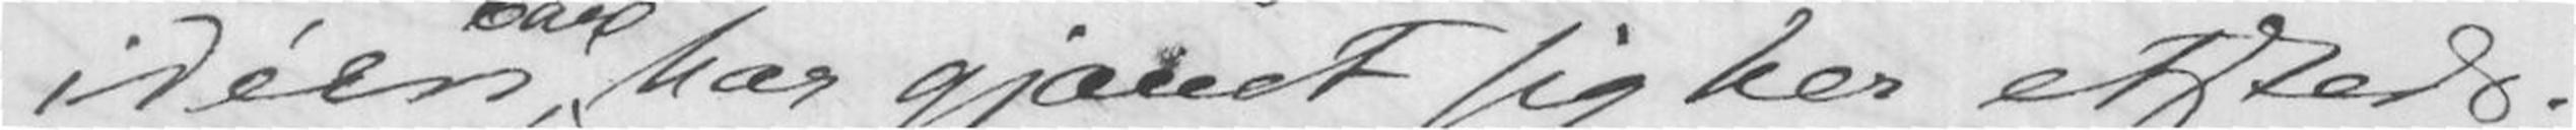ideen bare, har gjæmt sig her etsteds.
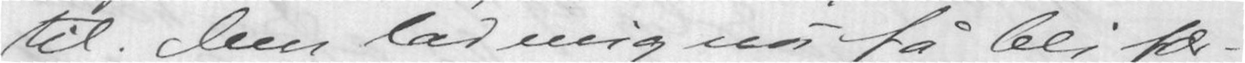til. Men lad mig nå få bli fær-
